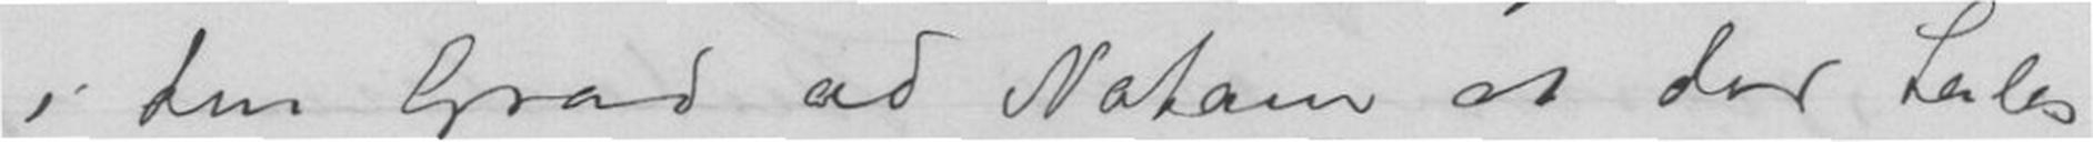i den Grad ad Notaen at der tales
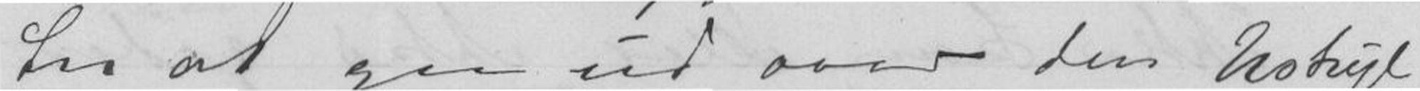til at gaa ud over den Uskul-
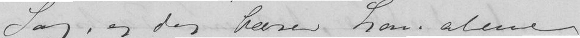Sag, og dog bærer han alene
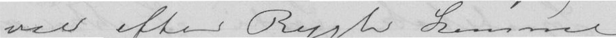vil efter Rygte komme
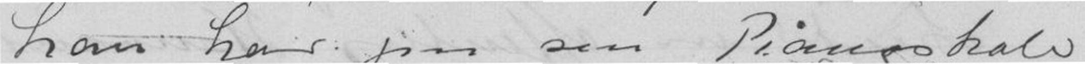han har paa sin Pianoskole
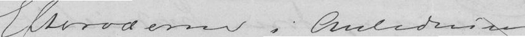Efteroderne i Anledning
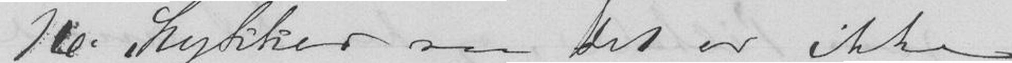16 Stykker saa det er ikke
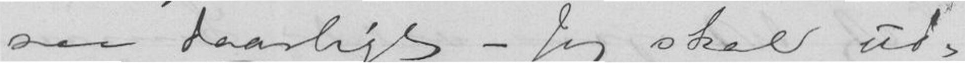saa daarligt - Jeg skal ud-
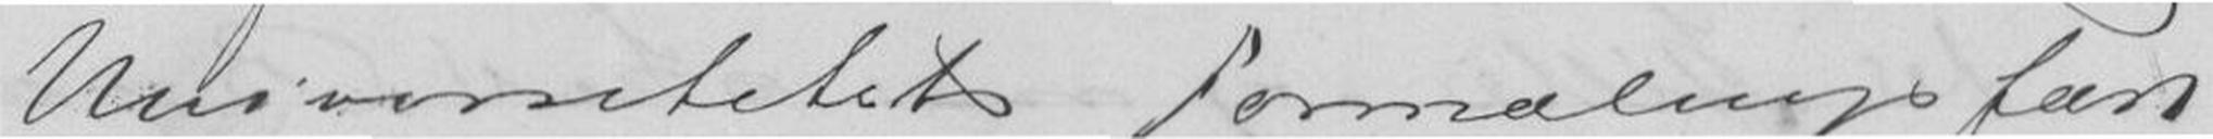Universitetets Formalingsfæst
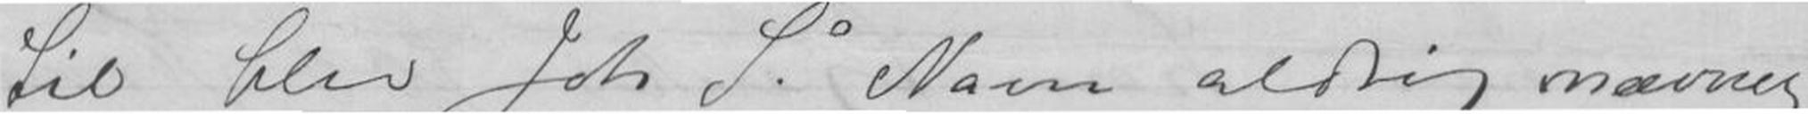til blir Joh Ss. Navn aldrig nævnet
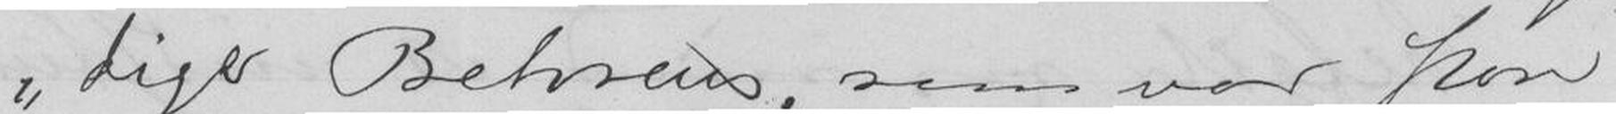dige Behrens, som vor store
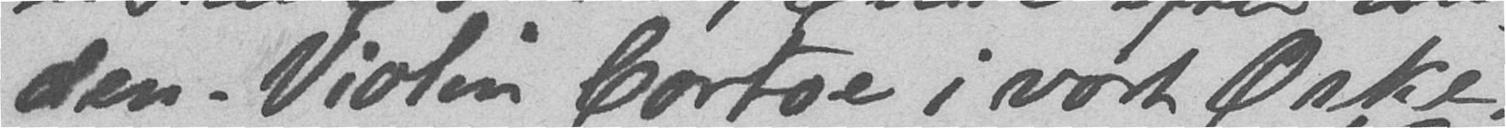den-Violin Cortoe i vort Orke-
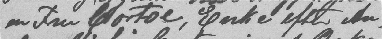en Fru Cortoe, Enke efter An-
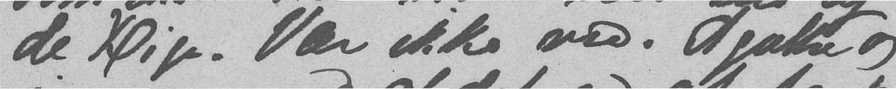de Rige. Vær ikke ved. Agathe og
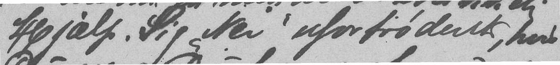Hjælp. Sig Nei ufortrødent, hvis
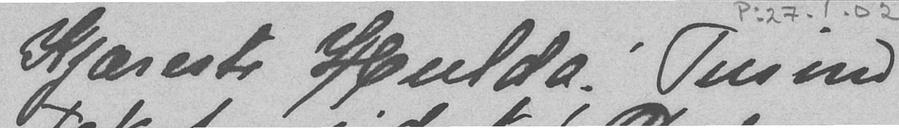Kjæreste Hulda. Tusind
